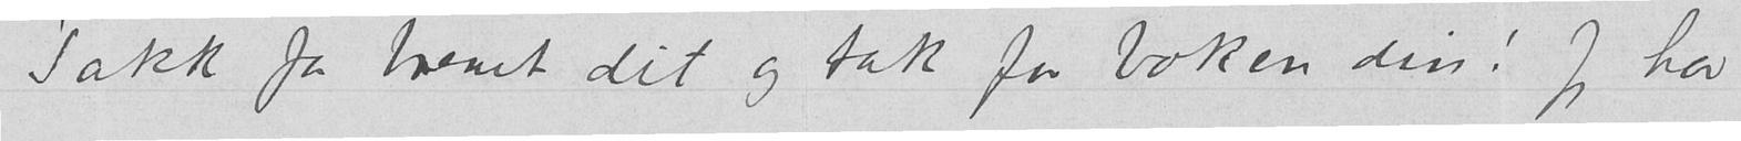Takk for brevet dit og tak for boken din! Jeg har
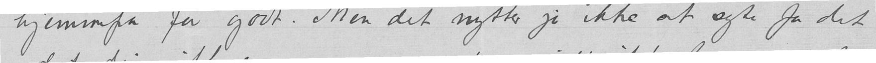hjemmefa for godt. Men det nytter jo ikke at syte for det
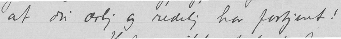at du ærlig og redelig har fortjent!
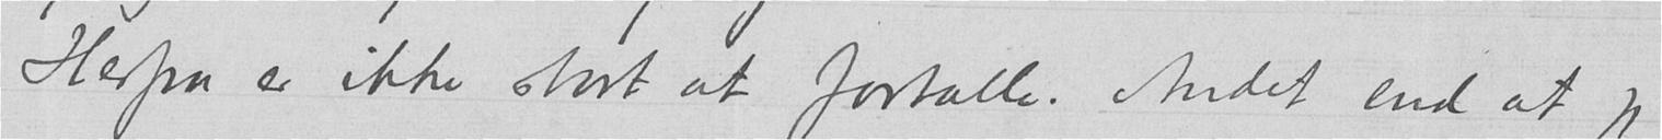Herfra er ikke stort at fortælle. Andet end at jeg
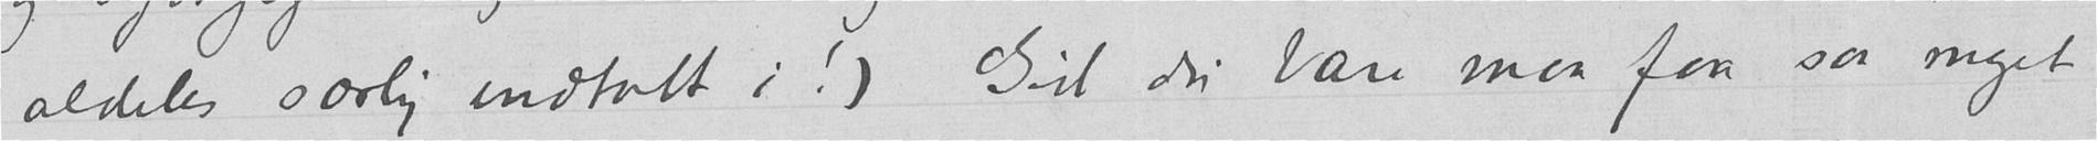aldeles særlig indtatt i!) Gid du bare maa faa saa meget
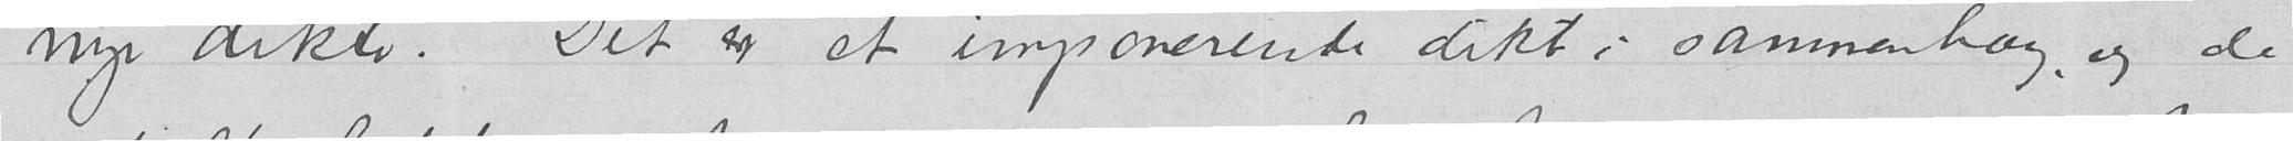nye dikte." Det er et imponerende dikt i sammenhæng, og de
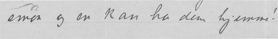smaa og en kan ha dem hjemme!
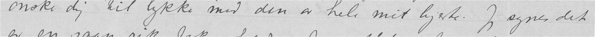ønske dig til lykke med den av hele mit hjerte. Jeg synes det
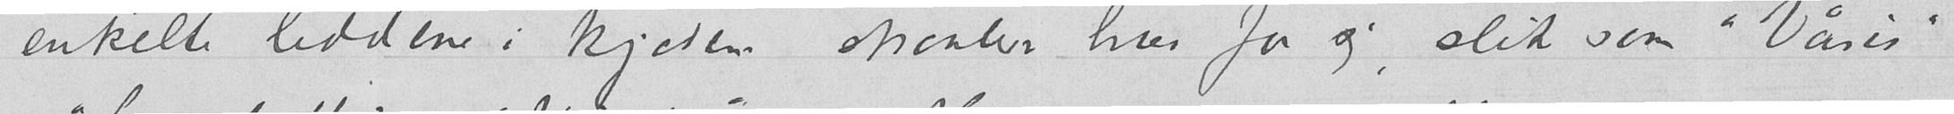enkelte leddene i kjeden straaler hver for sig, slik som "Våris"
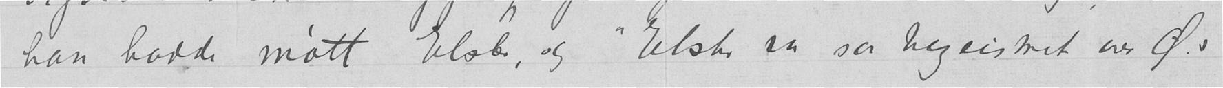han hadde møtt Elster, og "Elster var saa begeistret over Ø.s
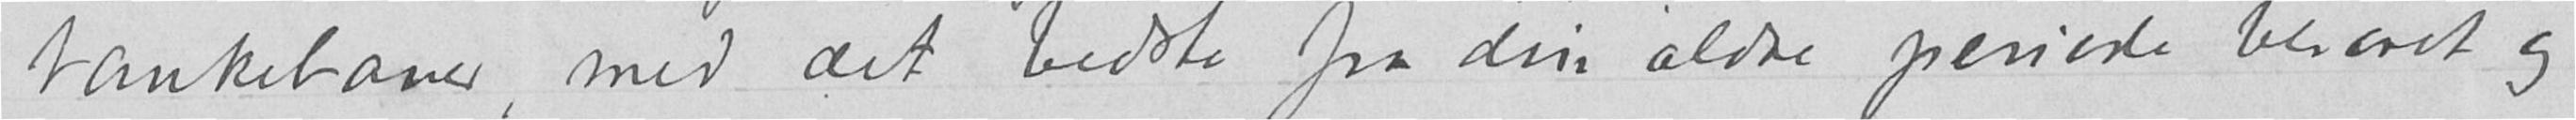tankebaner, med det bedste fra din ældre periode bevaret og
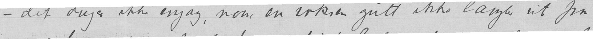– det duger ikke engang, naar en voksen gutt ikke længter ut fra
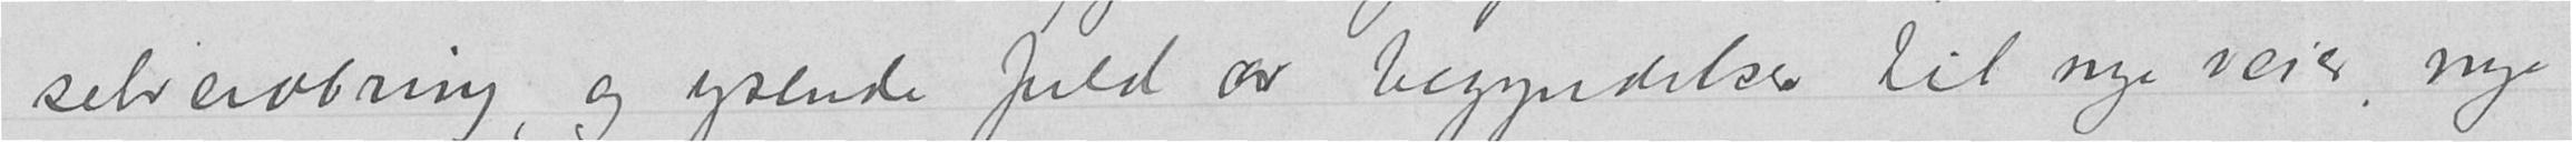selverobring, og yrende fuld av begyndelser til nye veier, nye
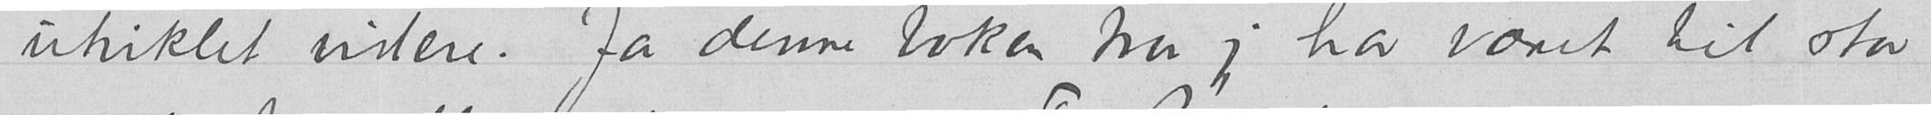utviklet videre. Ja denne boken tror jeg har været til stor
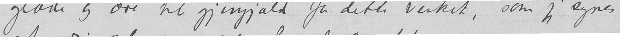glæde og ære til gjengjæld for dette verket, som jeg synes
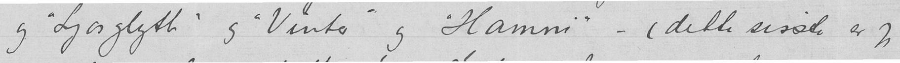og "Ljosglytt" og "Vinter" og "Hamni" – (dette sisste er jeg
